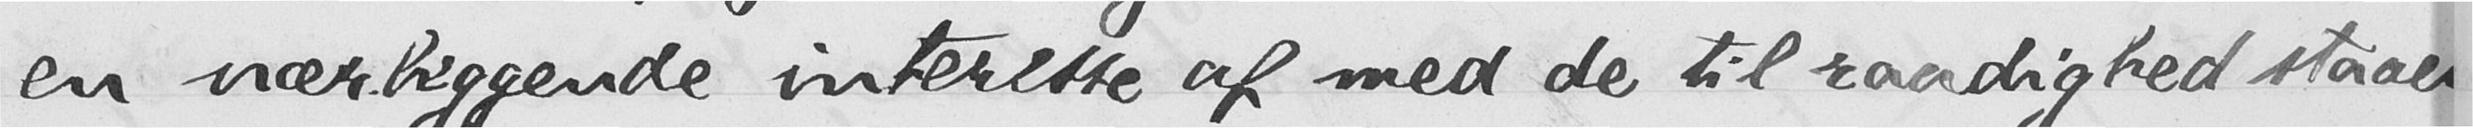en nærliggende interesse af med de til raadighed staa
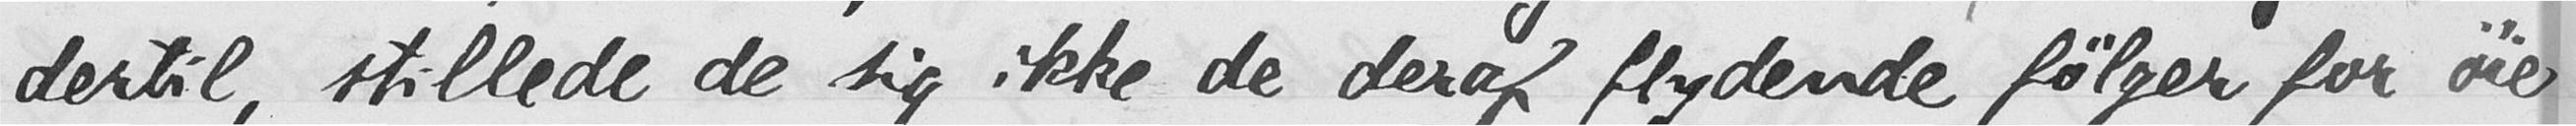dertil, stillede de sig ikke de deraf flydende følger for øie
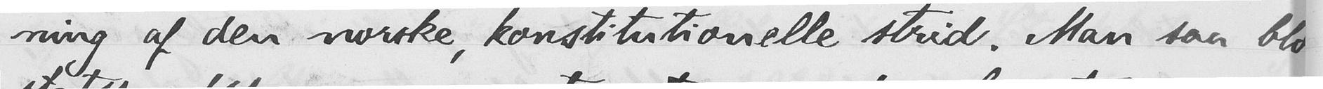ning af den norske, konstitutionelle strid. Man saa bl
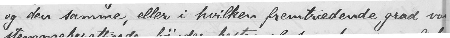og den samme, eller i hvilken fremtrædende grad vore
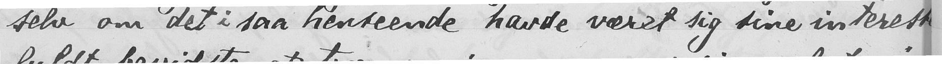selv om det i saa henseende havde været sig sine interess
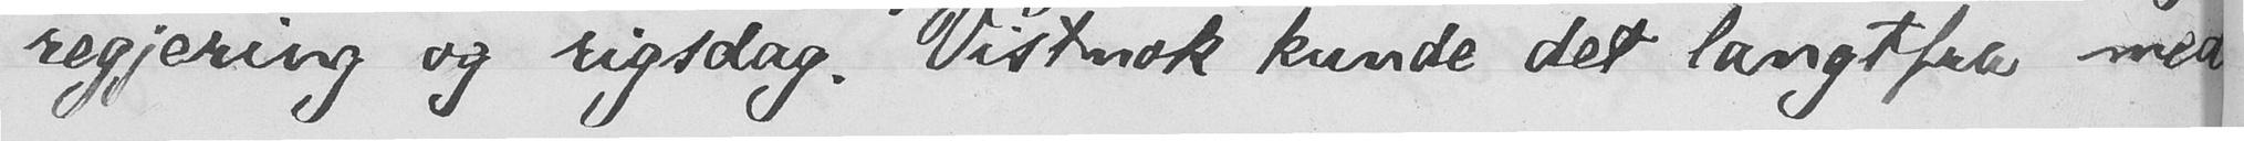regjering og rigsdag. Vistnok kunde det langtfra me
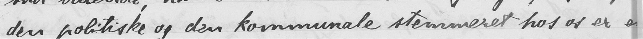den politiske og den kommunale stemmeret hos os er en
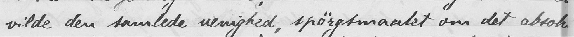vilde den samlede uenighed, spørgsmaalet om det absolute
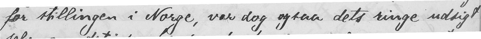for stillingen i Norge, var dog ogsaa dets ringe udsigt
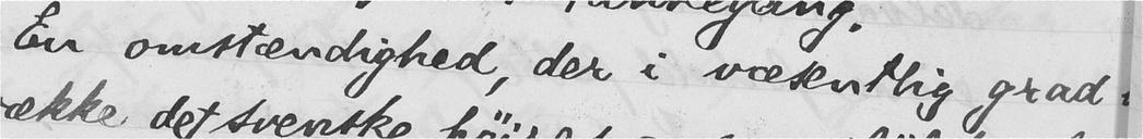En omstændighed, der i væsentlig grad bi-
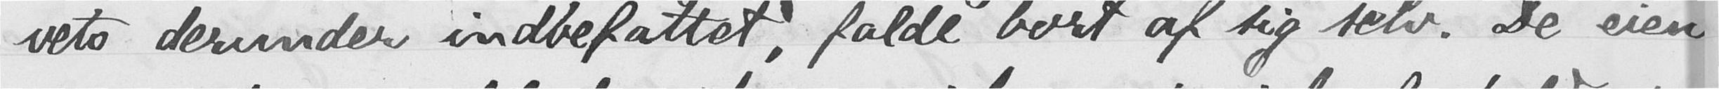veto derunder indbefattet, falde bort af sig selv. De eien-
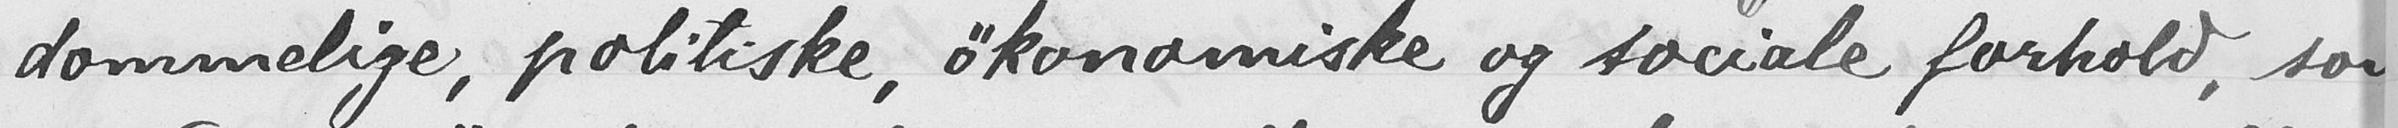dommelige, politiske, økonomiske og sociale forhold, som
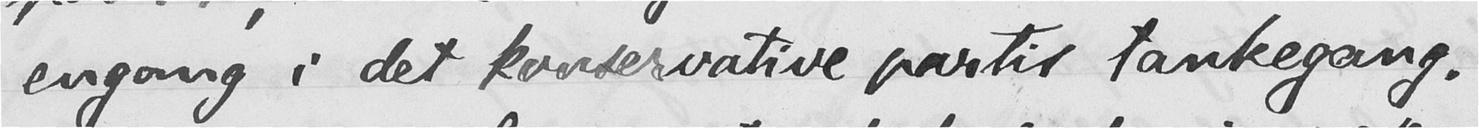engang i det konservative partis tankegang.
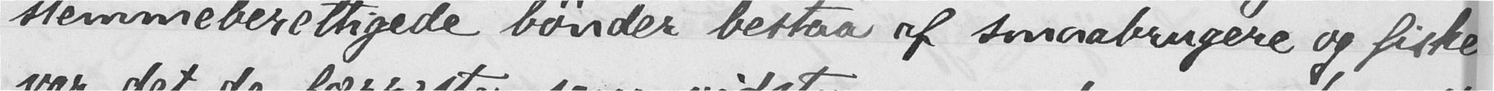stemmeberettigede bønder bestaa af smaabrugere og fiskere
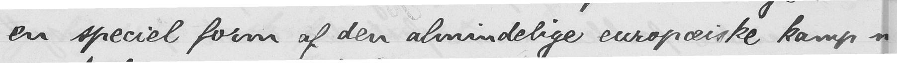en speciel form af den almindelige europæiske kamp
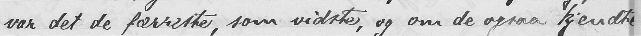var det de færreste, som vidste, og om de ogsaa kjendte
